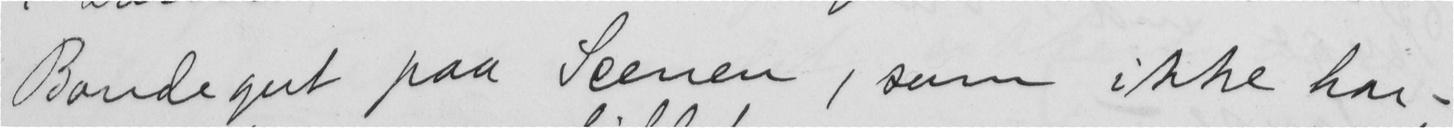Bondegut paa Scenen, som ikke har -
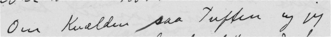Om Kvælden saa Tuften og jeg
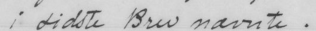i sidste Brev nævnte.
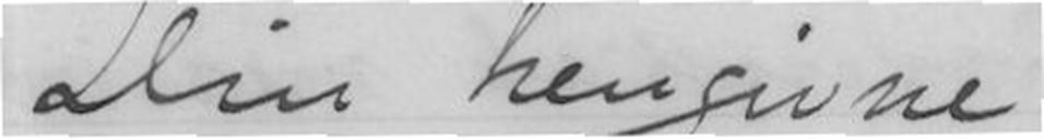Din hengivne
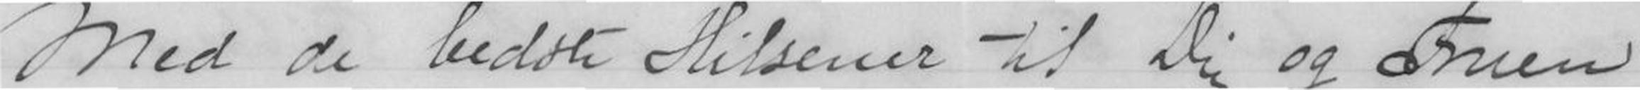Med de bedste Hilsener til Dig og Fruen
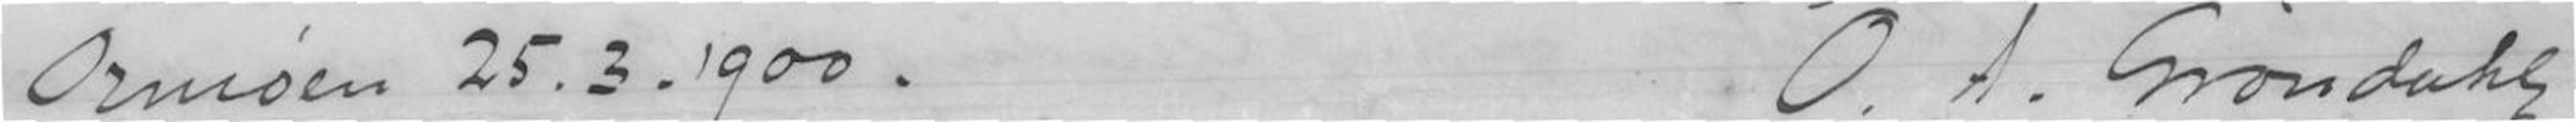O.A.Grøndahl Ormøen 25.3.1900.
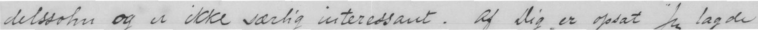delssohn og er ikke særlig interessant. Af Dig er opsat "Jeg lagde
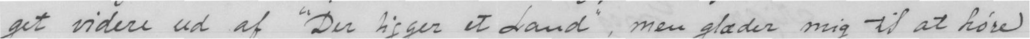get videre ud af "Der ligger et Land", men glæder mig til at høre
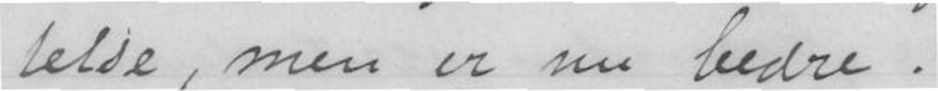lelse, men er nu bedre.
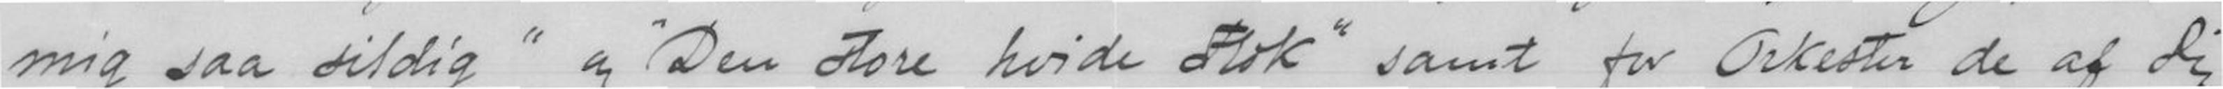mig saa tidlig" og Den store hvide Flok" samt for Orkester de af dig
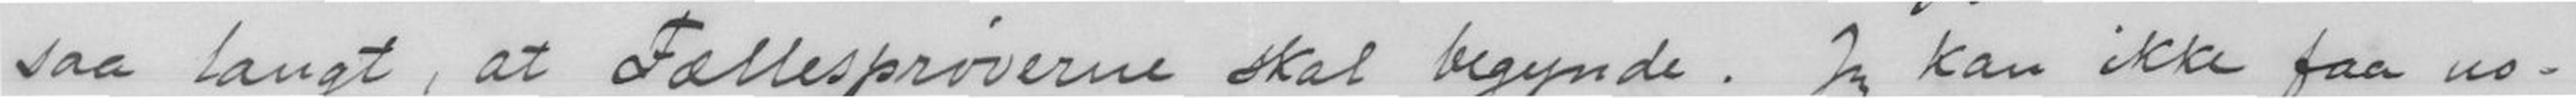saa langt, at Fællesprøvene skal begynde. Jeg kan ikke faa no-
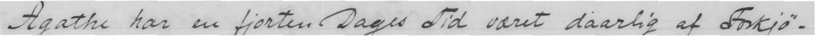Agathe har en fjorten Dagers Tid været daarlig af Forkjø-
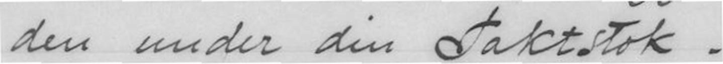den under din Taktstok.
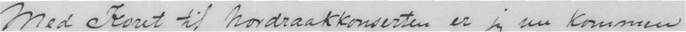Med Koret til Nordraakkonserten er jeg nu Kommen
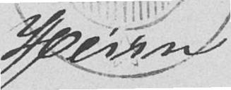Herr
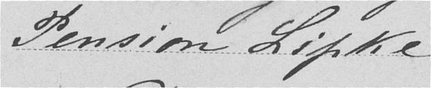Pension Lipke
